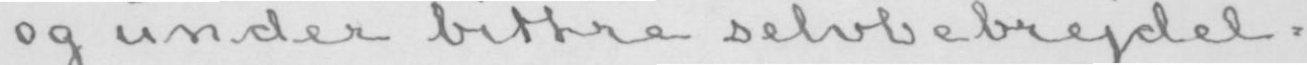og under bittre selvbebrejdel-
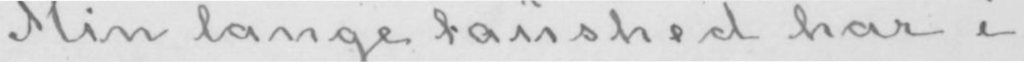Min lange taushed har i
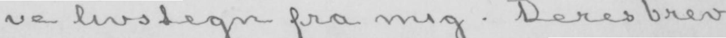ve livstegn fra mig. Deres brev
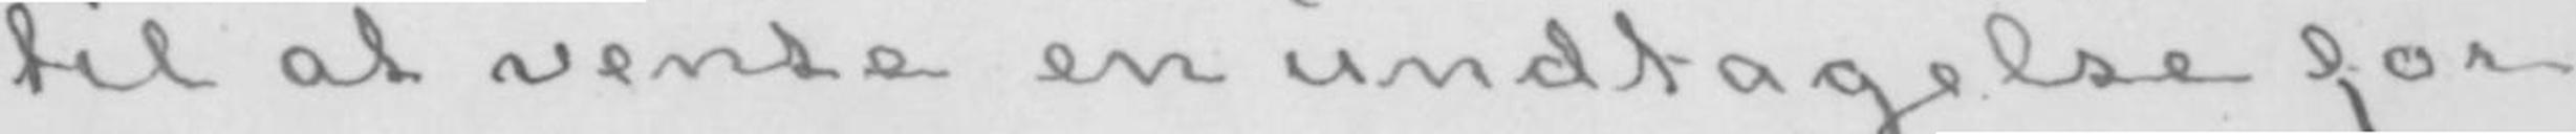til at vente en undtagelse for
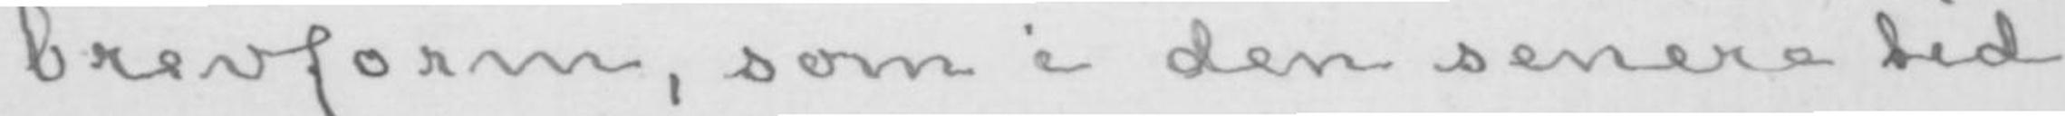brevform, som i den senere tid
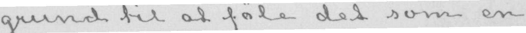grund til at føle det som en
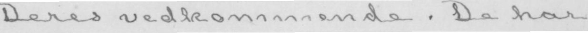Deres vedkommende. De har
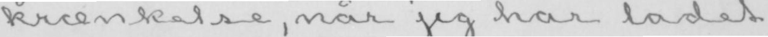krænkelse, når jeg har ladet
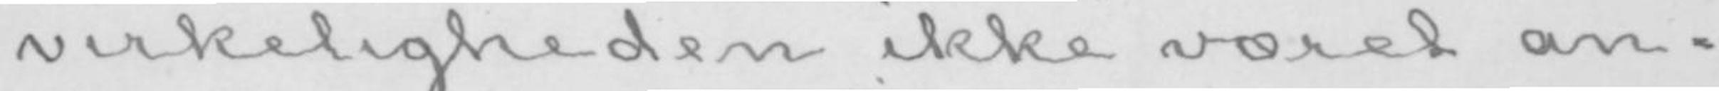virkeligheden ikke været an-
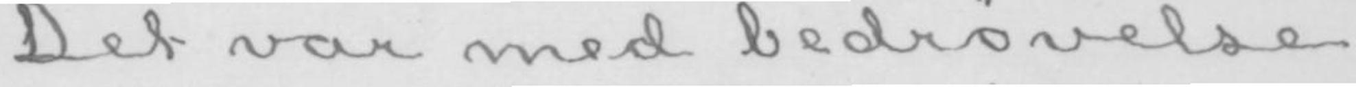Det var med bedrøvelse
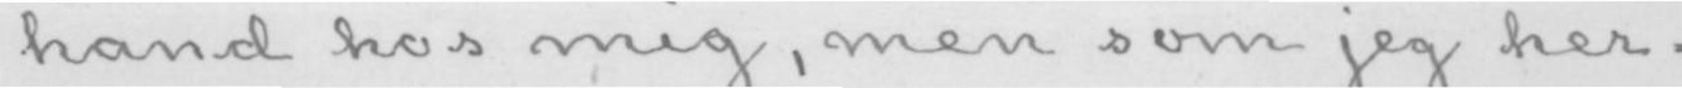hånd hos mig, men som jeg her-
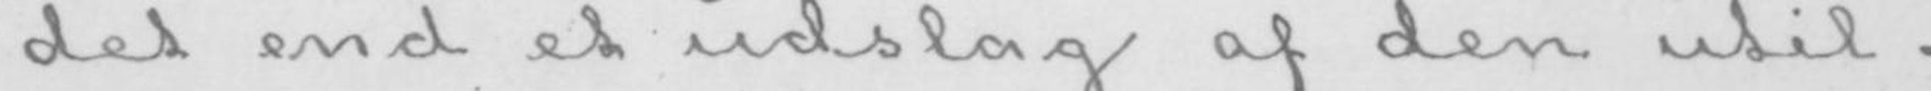det end et udslag af den util-
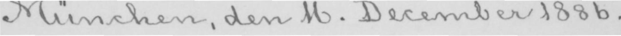München, den 16. December 1886.
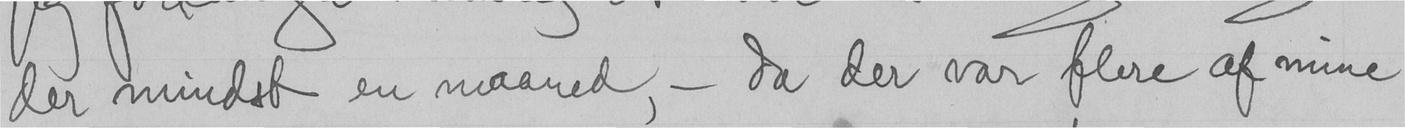der mindst en maaned - da der var flere af mine
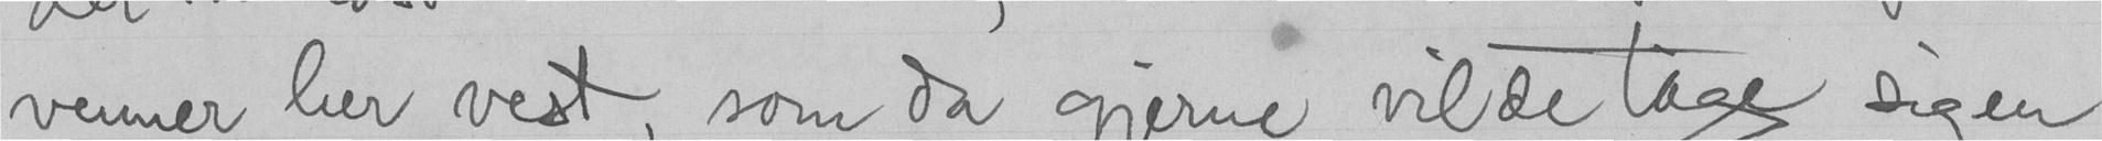venner her vest som da gjerne vilde tage sig en
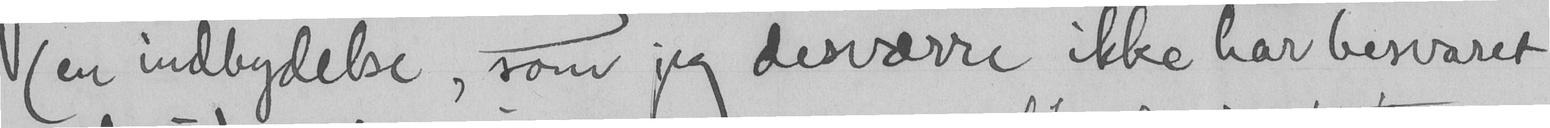(en indbydelse, som jeg desværre ikke har besvaret
